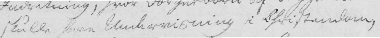skulle have Undervisning i Christendom,
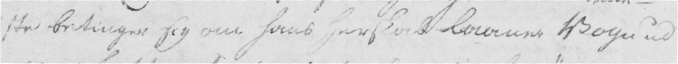ste betinger sig om hans Herskab laaner Vogn ud
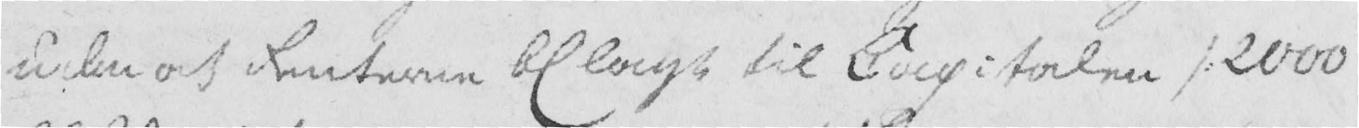uden at Renterne bl lagt til Capitalen /: 2000
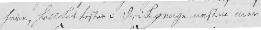have, hvilket koster i drickepenge nesten mer
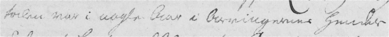talen var i nogle Aar i Arvingernes Hender
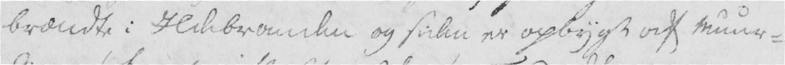brændte i Ildebranden og siden er opbygt af Muur-
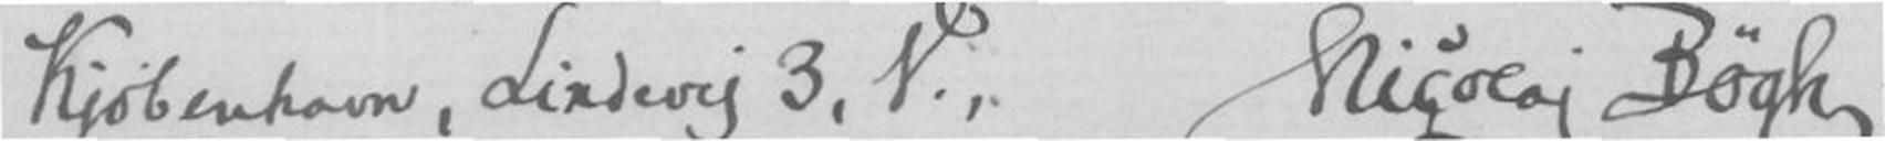Kjøbenhavn, Lindevej 3, V., Nicolai Bøgh
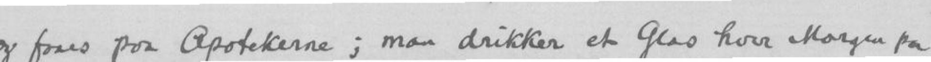og faaes paa Apotekerne; man drikker et glas hver Morgen paa
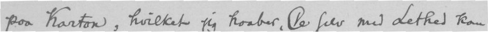paa Karton, hvilket jeg haaber De selv med Lethed kan
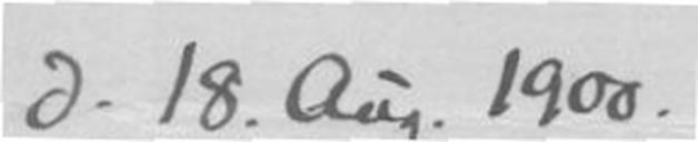D. 18. Aug 1900
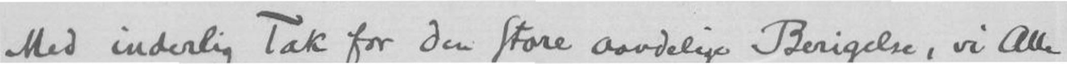Med inderlig Tak for den store aandelige Berigelse, vi Alle
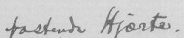fastende Hjærte.
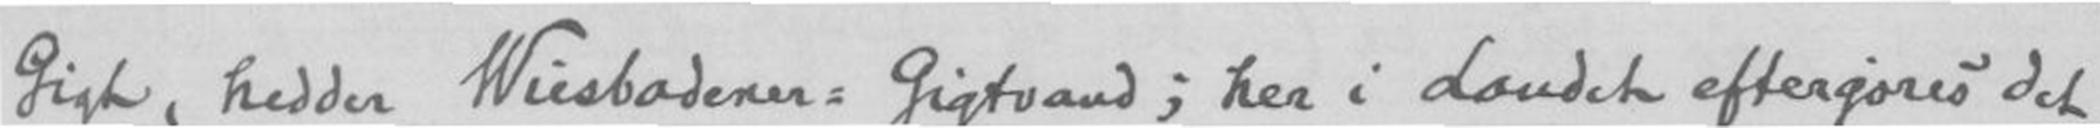Gigt, hedder Wiesbadener-Gigtvand; her i Landet eftergøres det
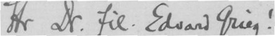Hr Dr. fil. Edvard Grieg!
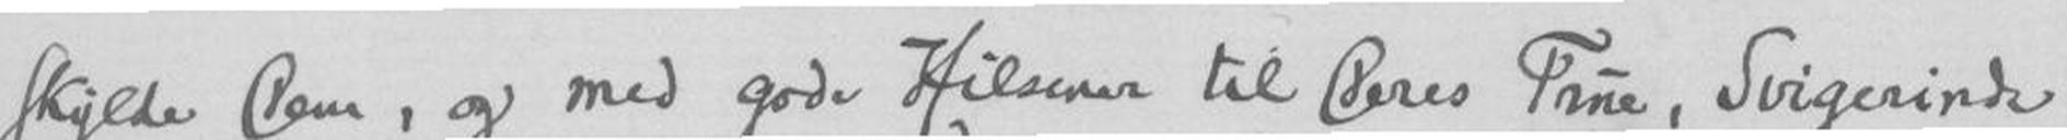skylte Dem, og med gode Hilsener til Deres Frue, Svigerinde
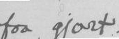faa gjort.
